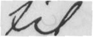til
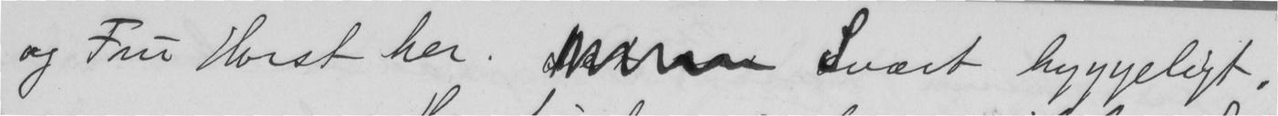og Fru Horst her.  Svært hyggeligt.
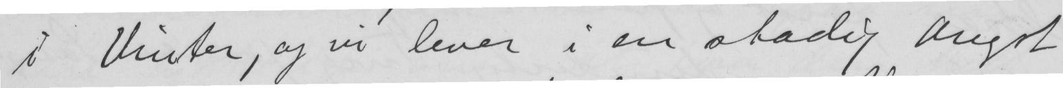i Vinter, og vi lever i en stadig Angst
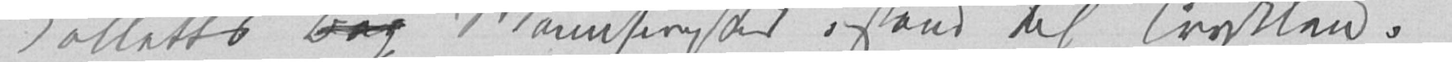Colletts Bog Manuscripter istand til Trykken.
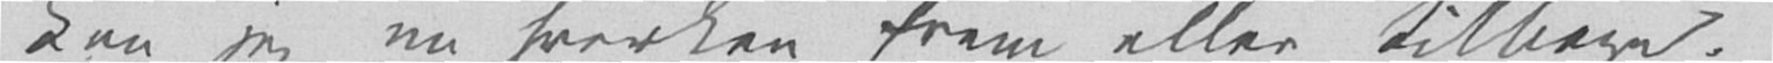kan jeg nu hverken frem eller tilbage.
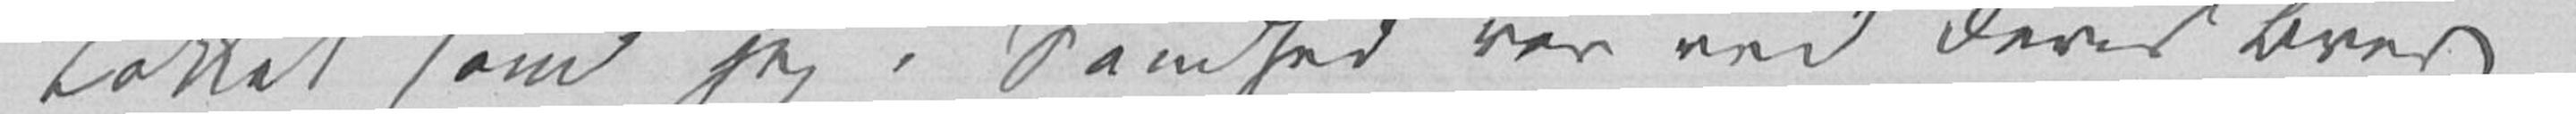Lokket som jeg i Sandhed var ved Deres Brev
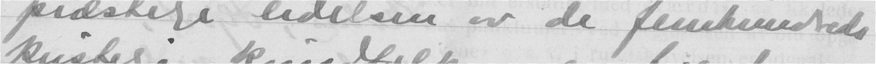præstelige ledelsen av de femhundrede
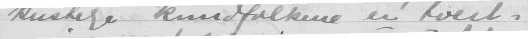kristelige kvindfolkene er tvert-
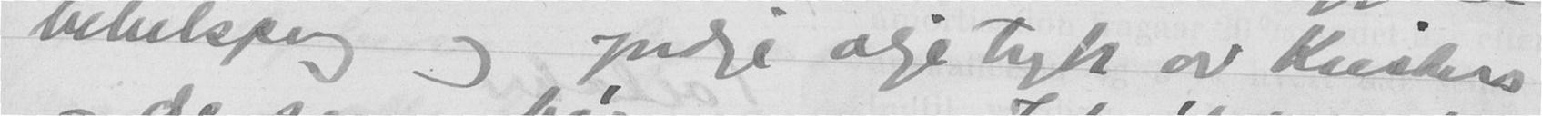bibelsprog og yndige ulige tryk av Kristus
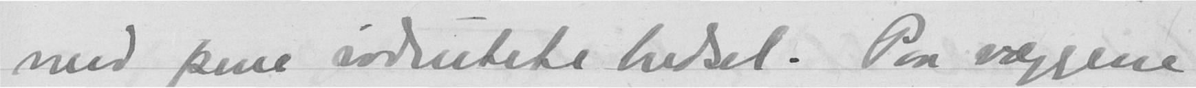med pene rødrutete hedsel. Paa væggene
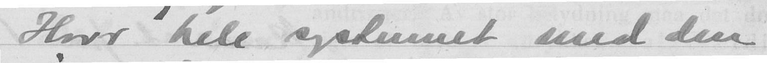Hvor hele systemet med den
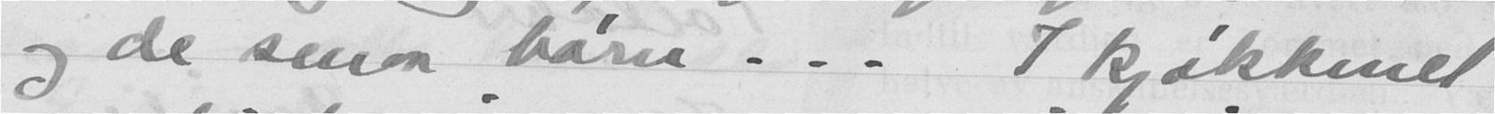og de smaa børn... I kjøkkenet
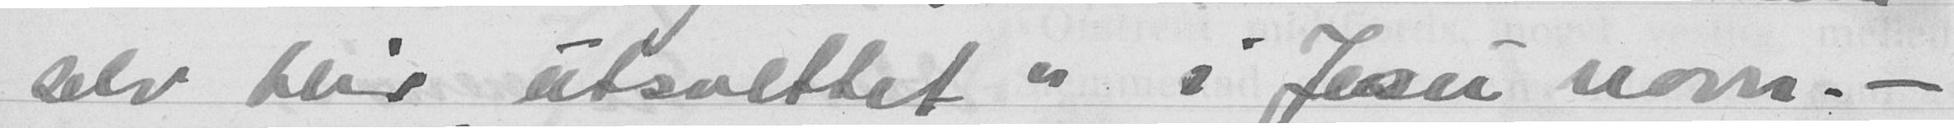selv blir "utsvettet" i Jesu navn. -
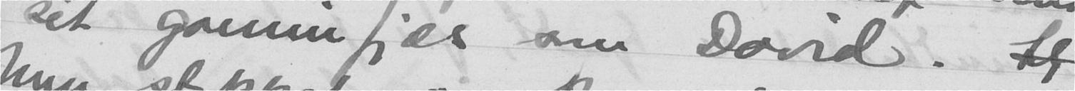sit gummifjæs som David. Jt
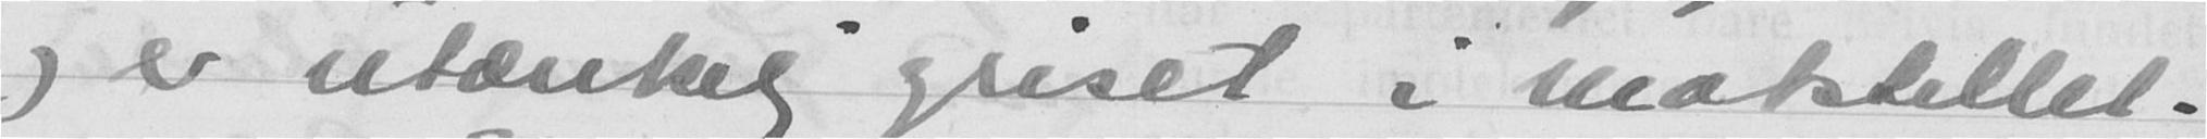og er utænkelig griset i matstellet.
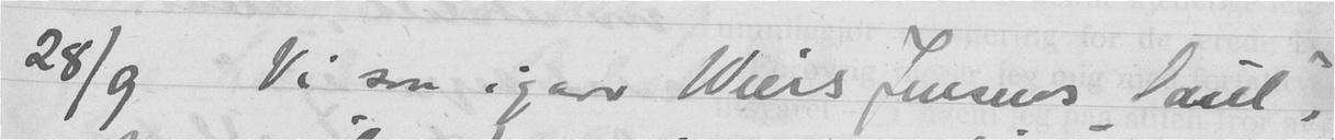28/9 Vi saa igaar Wiers Jensens Saul,
